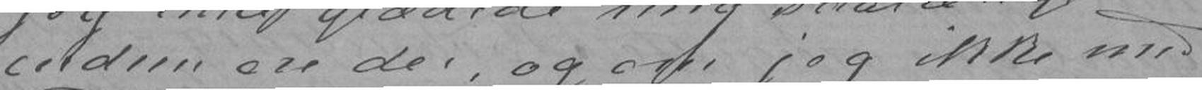endnu ere der, og om jeg ikke med
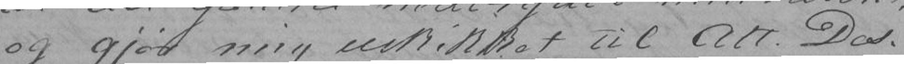og gjør mig uskikket til Alt. Des-
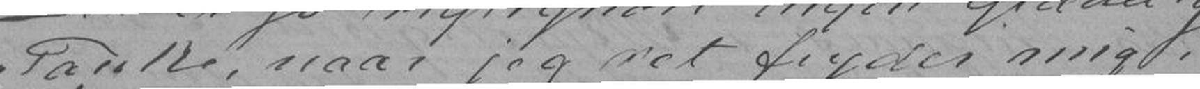Tanke, naar jeg ret fryder mig i
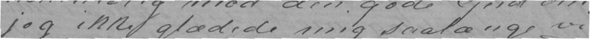jeg ikke glædede mig saalænge vi
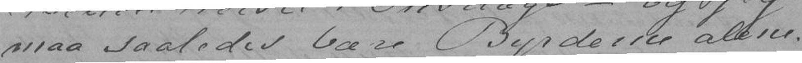maa saaledes bære Byrderne alene.
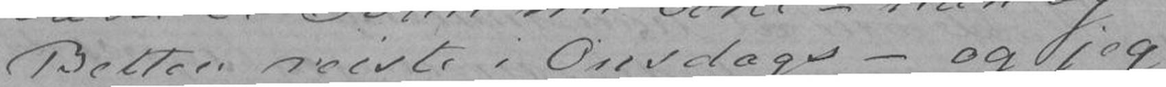Betten reiste i Onsdags - og jeg
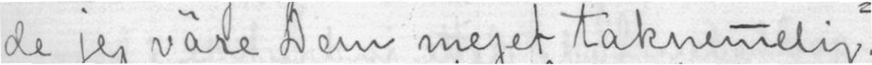de jeg väre Dem meget taknemlig.
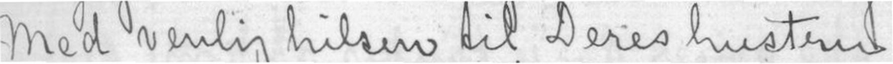Med venlig hilsen til Deres hustru
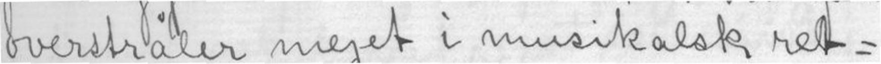overstråler meget i musikalsk ret-
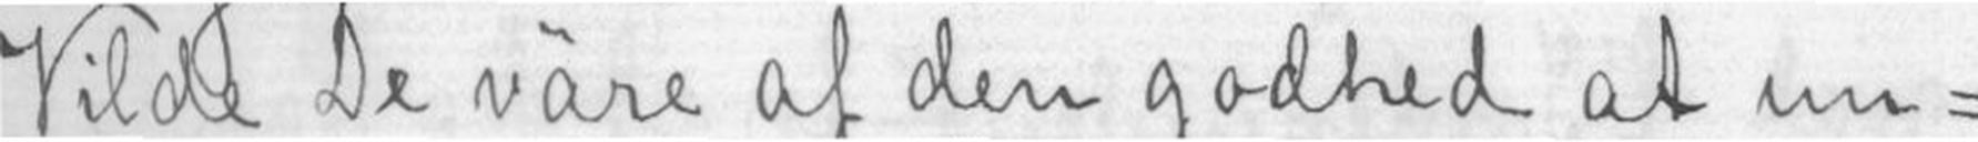Vilde De väre af den godhed at un-
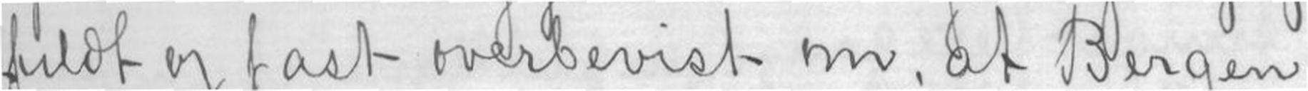fuldt og fast overbevist om, at Bergen
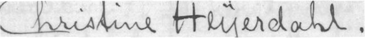Christine Heyerdahl.
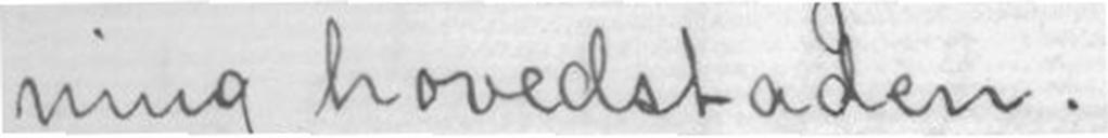ning hovedstaden. -
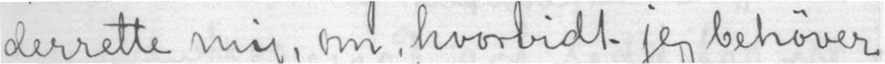derrette mig, om, hvorvidt jeg behøver
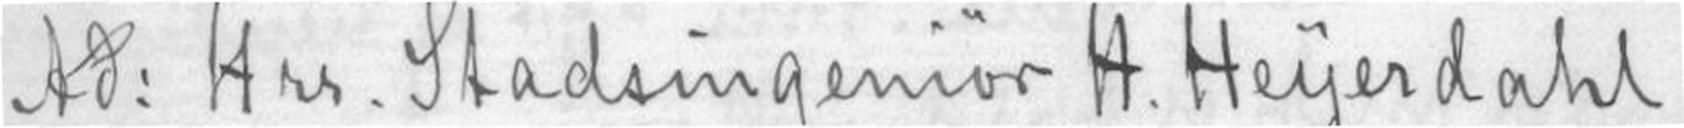Ad: Hrr. Stadsingeniör H. Heyerdahl
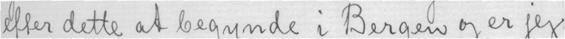efter dette at begynde i Bergen og er jeg
